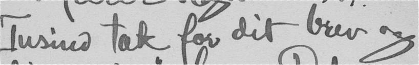Tusind tak for dit brev og
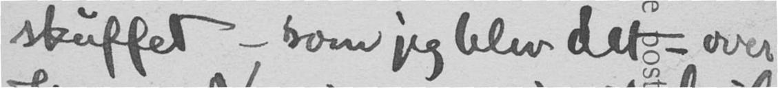skuffet - som jeg blev det - over
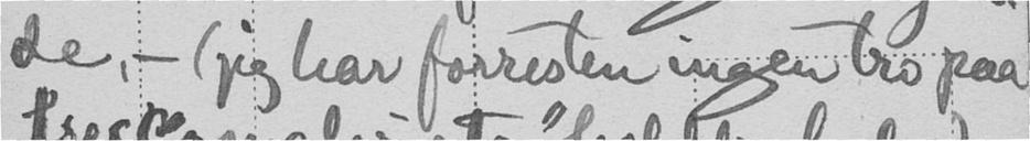de, - (jeg har forresten ingen tro paa
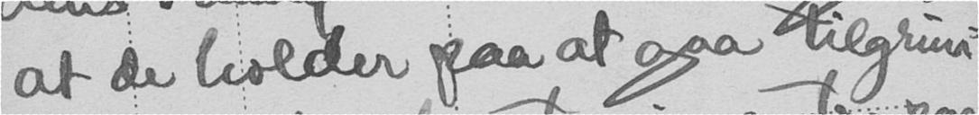at de holder paa at gaa tilgrun-
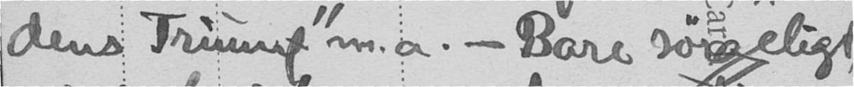dens Triumf" m.a. - Bare sørgeligt,
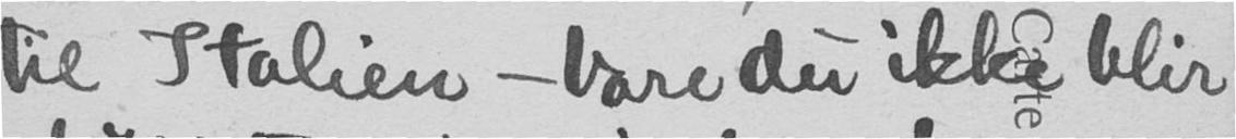til Italien - bare du ikke blir
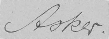Asker.
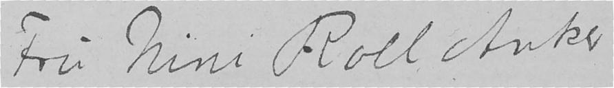Fru Nini Roll Anker
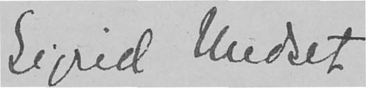Sigrid Undset
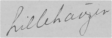Lillehaugen
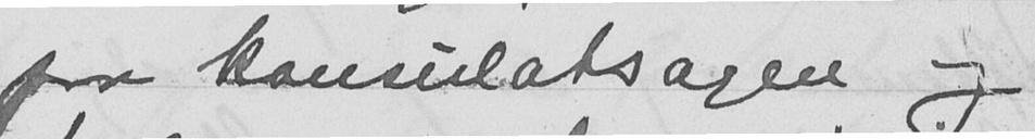paa konsulatsagen og
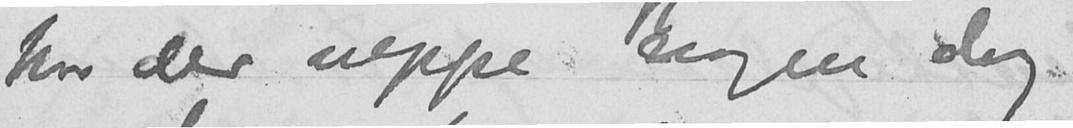har der neppe nogen dag
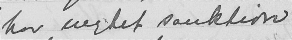har negtet sanktion
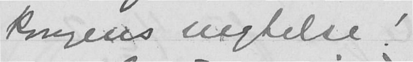kongens negtelse!
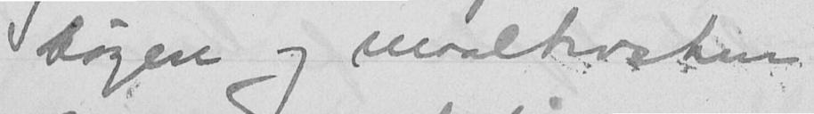bøgen og maaltrosten
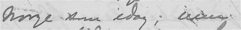Norge som idag; men
